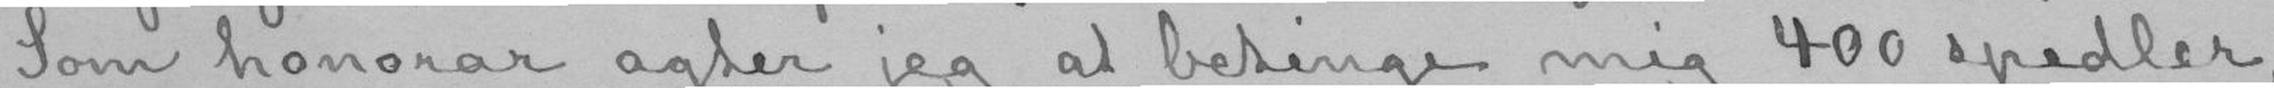Som honorar agter jeg at betinge mig 400 spedler,
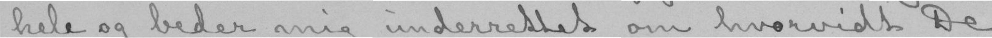hele og beder mig underrettet om hvorvidt De
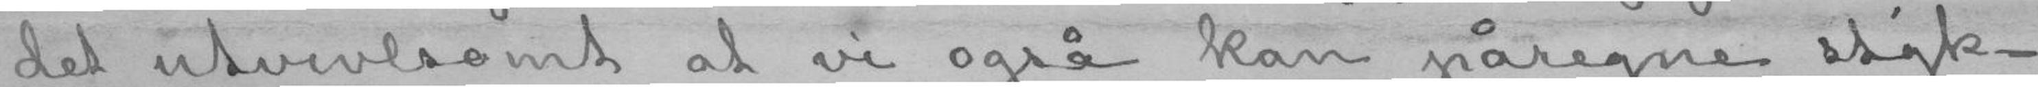det utvivlsomt at vi også kan påregne styk-
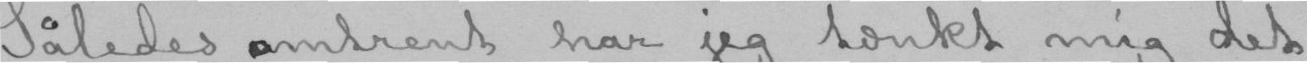Således omtrent har jeg tænkt mig det
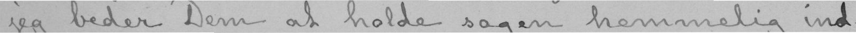jeg beder Dem at holde sagen hemmelig ind-
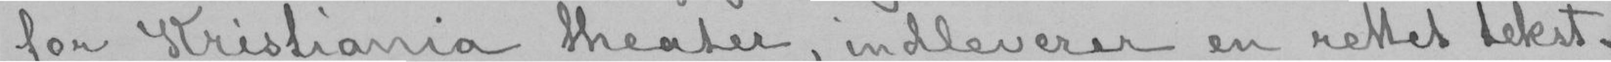for Kristiania theater, indleverer en rettet tekst-
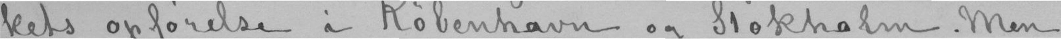kets opførelse i København og Stokholm. Men
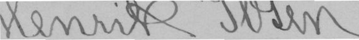Henrik Ibsen
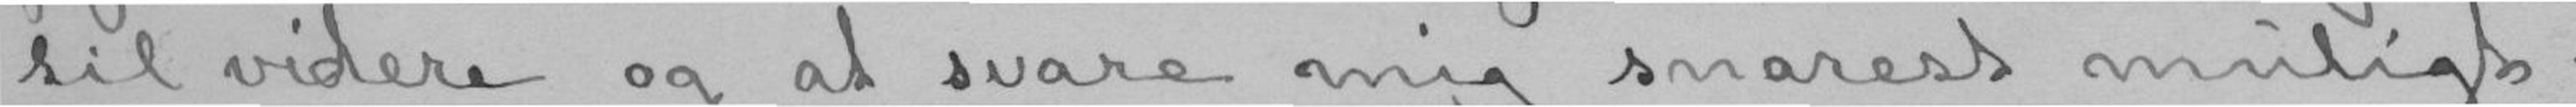til videre og at svare mig snarest muligt.
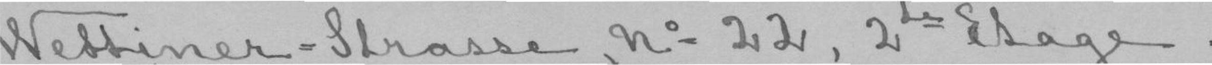Wettiner-Strasse, N. 22, 2den Etage.
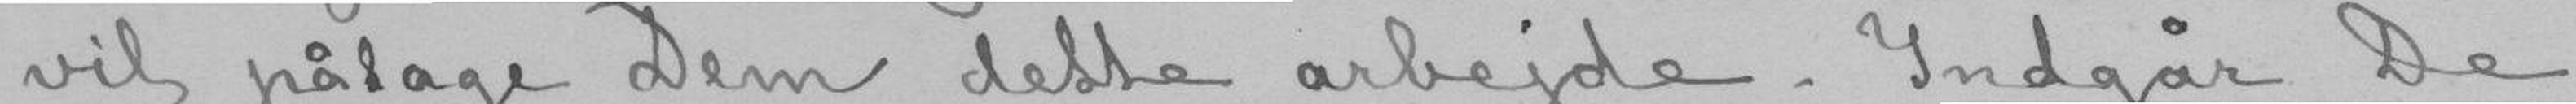vil pålage Dem dette arbejde. Indgår De
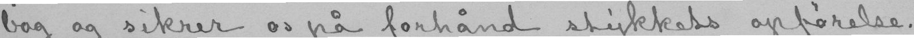bog og sikrer os på forhånd stykkets opførelse.
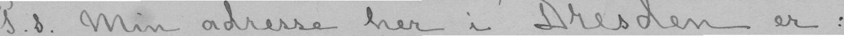P.s Min adresse her i Dresden er:
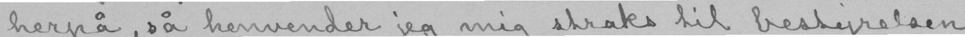herpå, så henvender jeg mig staks til bestyrelsen
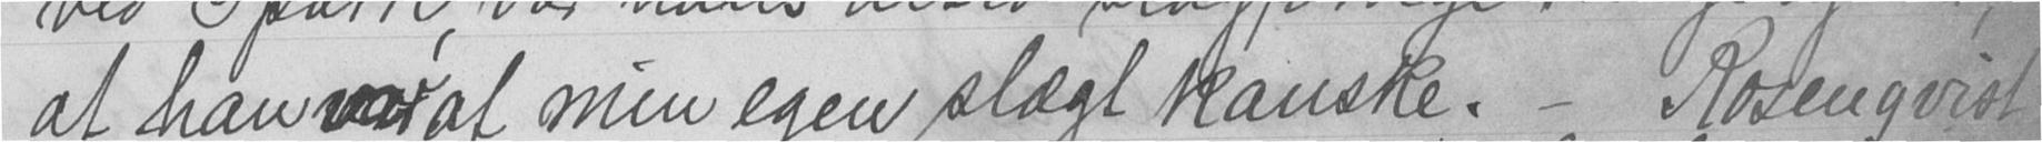at han var at min egen slægt kanske. - Rosenqvist
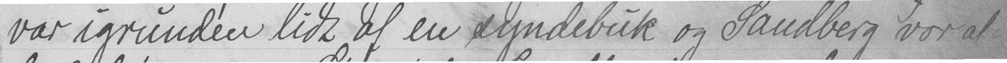var igrunden lidt af en syndebuk og Sandberg vor al-
